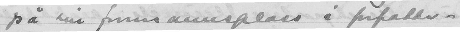på sin formannsplass i forfatte-
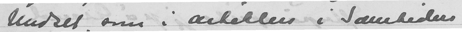Undset som i artiklen i Samtiden
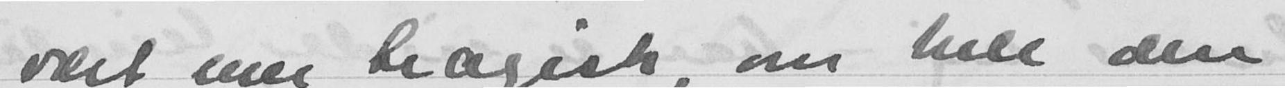vært mer tragisk, om hele den
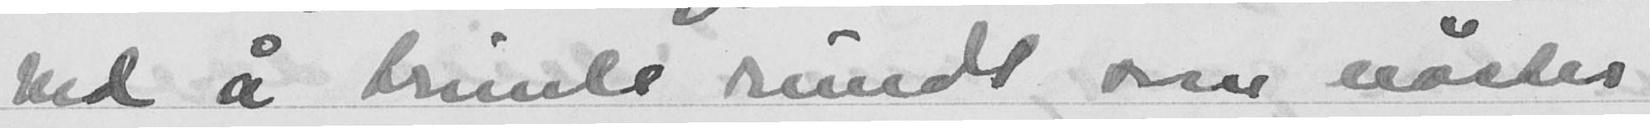ved å trimle rundt som nøster
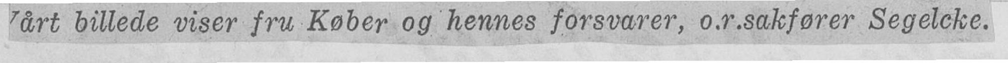Vårt billede viser fru Køber og hennes forsvarer, o.r.sakfører Segelcke.
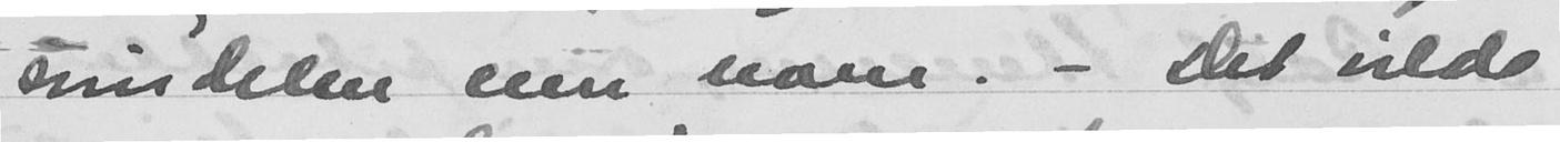sine deler enn noen. - Det vilde
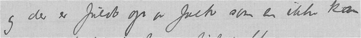og der er fuldt op av folk som en ikke kan
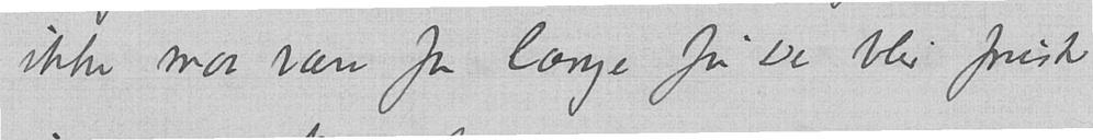ikke maa vare for længe før De blir frisk
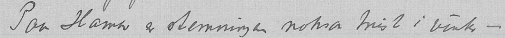Paa Hamar er stemningen noksaa trist i vinter –
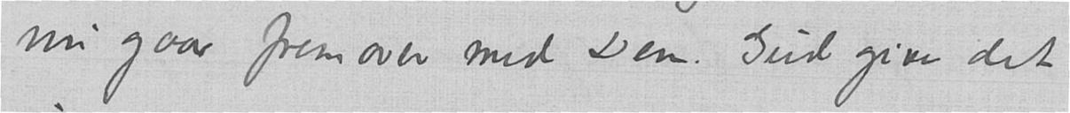nu gaar fremover med Dem. Gud give det
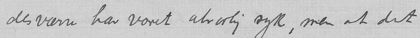desværre har været alvorlig syk, men at det
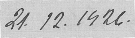21.12.1926.
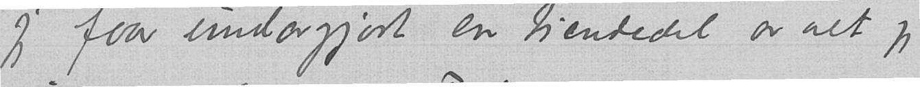jeg faar undagjort en tiendedel av alt jeg
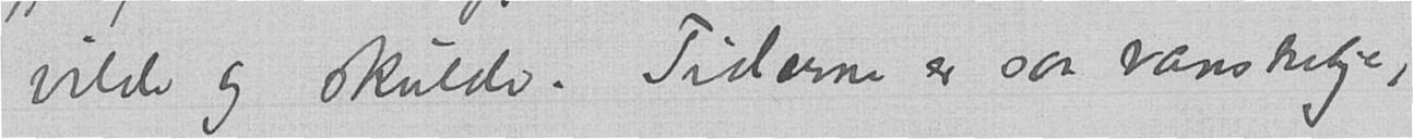vilde og skulde. Tiderne er saa vanskelige,
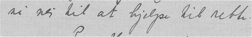si nei til at hjelpe til rette.
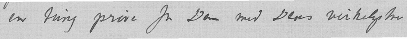en tung prøve for Dem med Deres virkelystne
